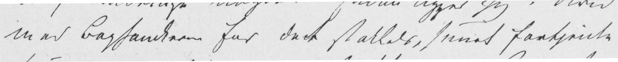med Boghandlerne for det stakkels, suurt fortjente
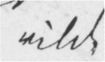vilde
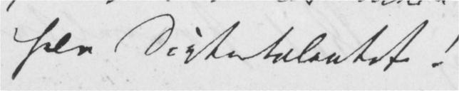hele Digtertalentet!
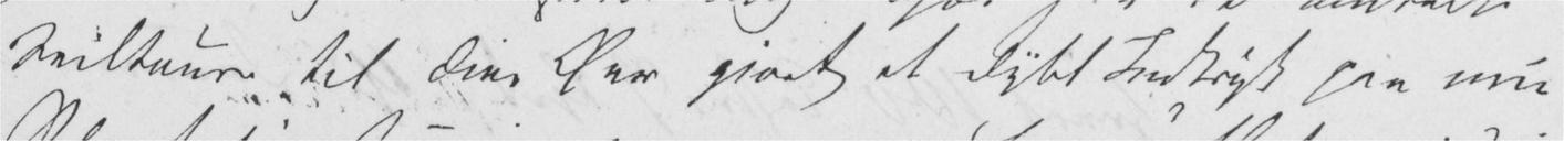Seiltoure til Dine Øer gjort et dybt Indtryk paa min
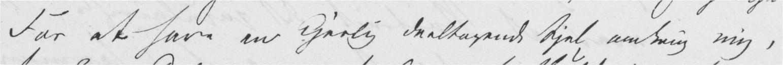For at have en kjerlig deeltagende Sjel omkring mig,
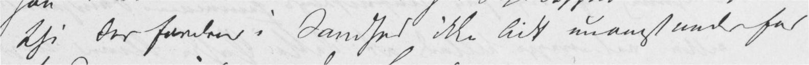thi der fordres i Sandhed ikke lidt nu omstunder for
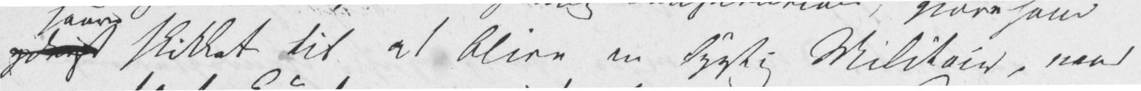yderst skikket til at blive en dygtig Militair, naar
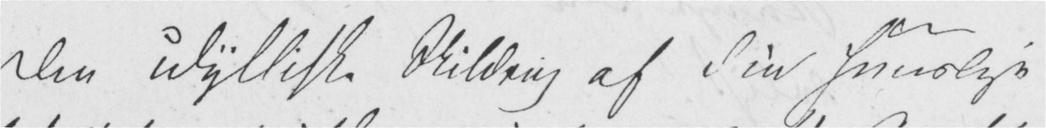Den idylliske Skildring af Din huuslige
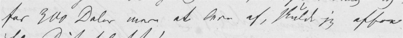for 300 Daler mere at leve af, skulde jeg afsee
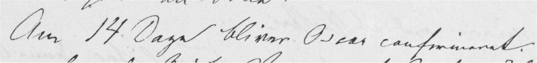Om 14 Dage bliver Oscar confirmeret.
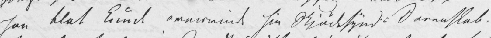han blot kunde overvinde sin Skjødesynd: Dovenskab.
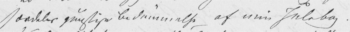særdeles gunstige Bedømmelse af min Julebog.
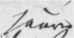saare
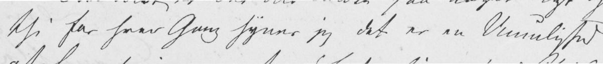thi for hver Gang synes jeg det er en Umulighed
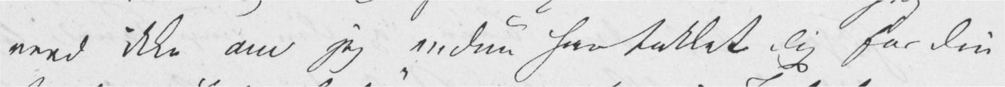veed ikke om jeg endnu har takket Dig for Din
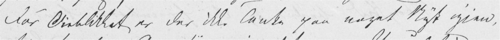For Øieblikket er der ikke Tanke paa noget Nyt igjen,
